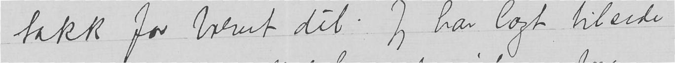takk for brevet dit. Jeg har lagt til side
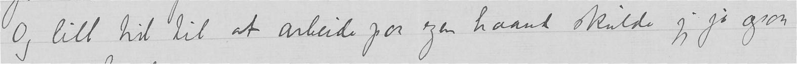Og litt tid til at arbeide paa egen haand skulde jeg jo ogsaa
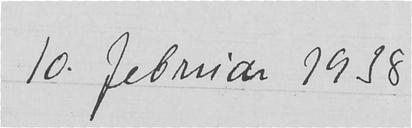10. februar 1938
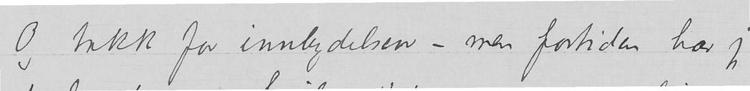Og takk for innlydelsen – men fortiden har jeg
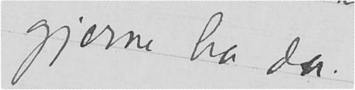gjerne ha da.
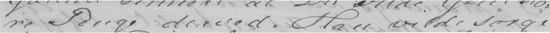re Penge derved. Han vilde sørge
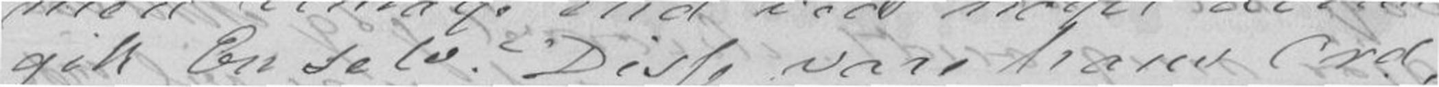gik En selv. Disse vare hans Ord,
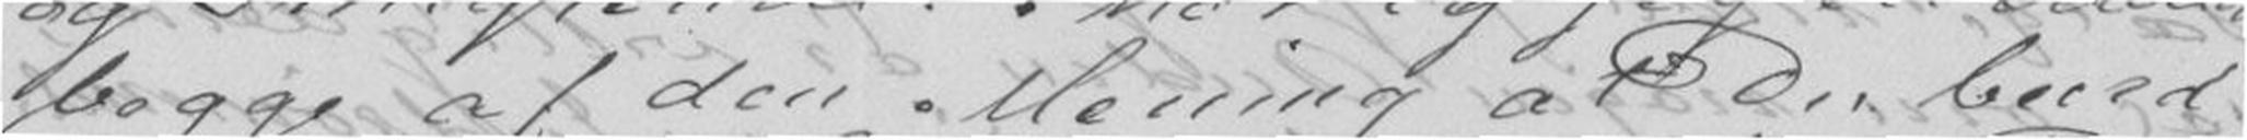begge af den Mening at Du burde
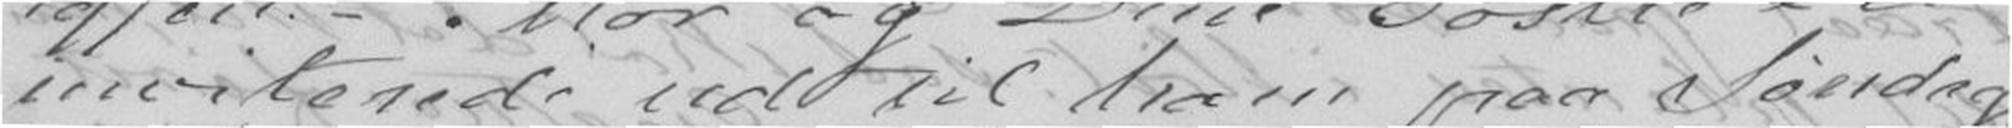inviterede ud til ham paa Søndag,
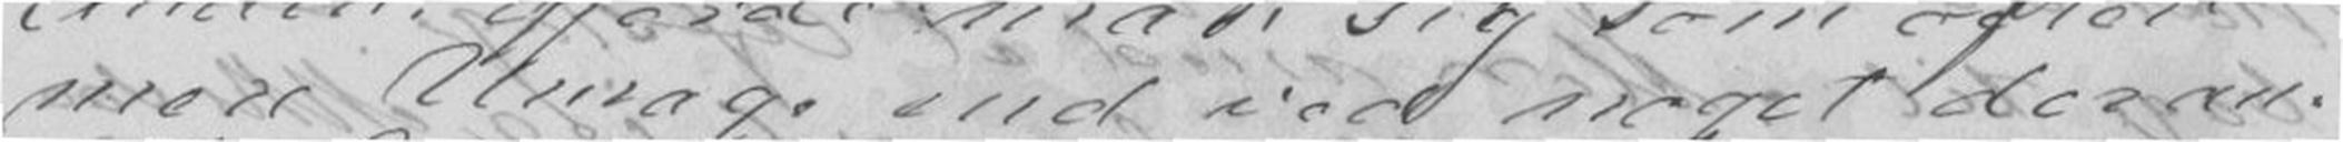mere Umage end ved noget der an-
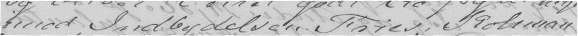imod Indbydelsen. Fries, Kohman
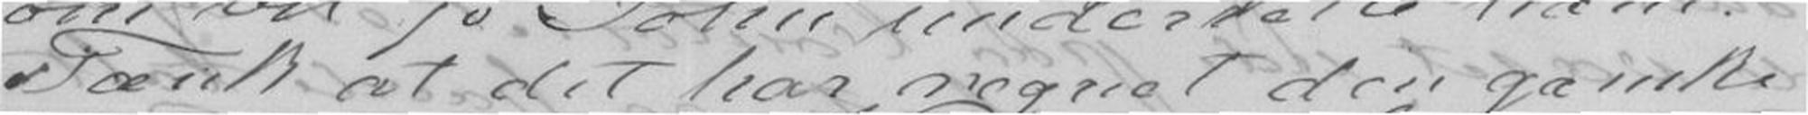Tænk at det har regnet den ganske
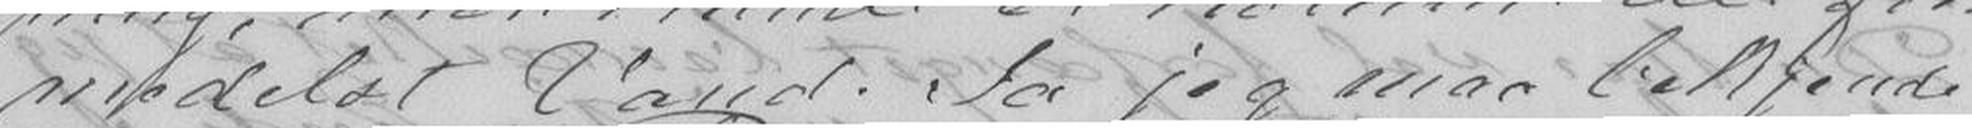medelst Vand. Ja jeg maa bekjende
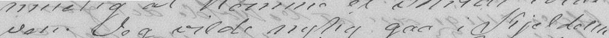ven. Jeg vilde nylig gaa i Kjelderne
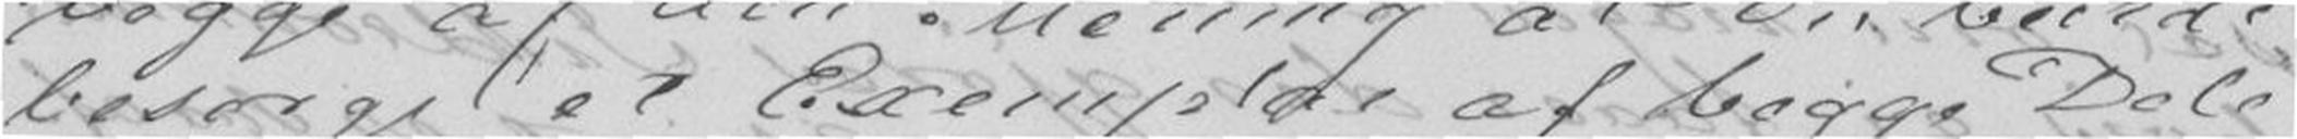besørge et Exemplar af begge Dele
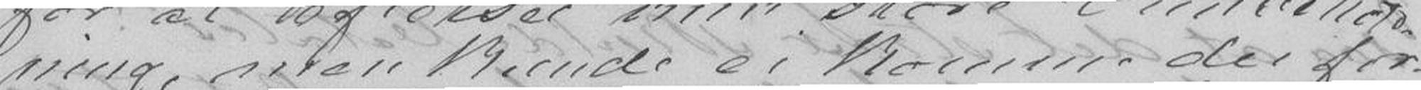ning, men kunde ei komme der for
